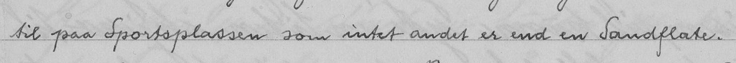til paa Sportsplassen som intet andet er end en Sandflate.
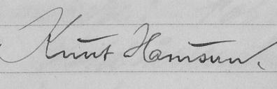Knut Hamsun.
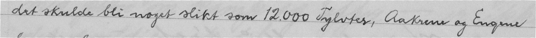det skulde bli noget slikt som 12.000 Tylvter, Aakrene og Engene
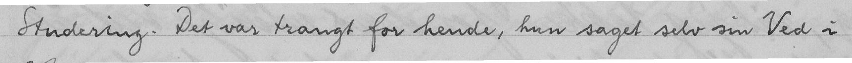Studering. Det var trangt for hende, hun saget selv sin Ved i
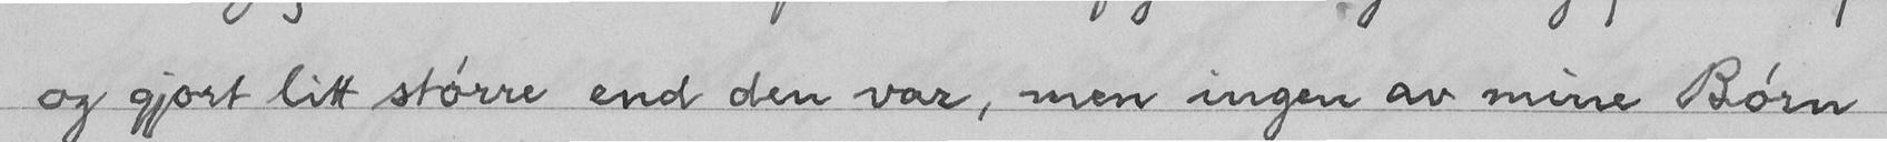og gjort litt større end den var, men ingen av mine Børn
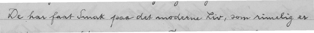De har faat Smak paa det moderne Liv, som rimelig er
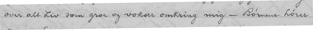over alt Liv som gror og vokser omkring mig - Børnene hører
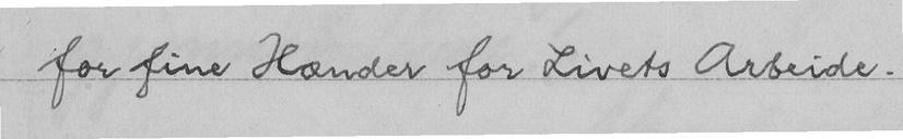for fine Hænder for Livets Arbeide.
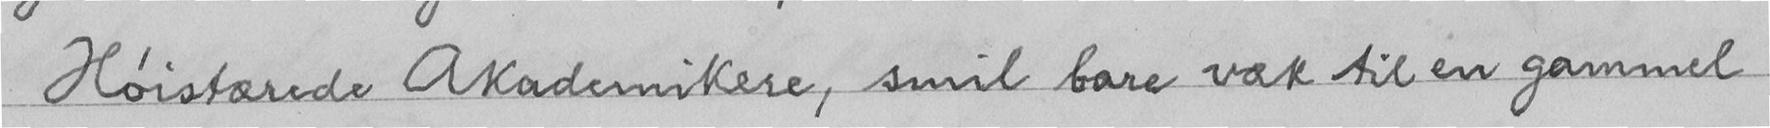Høistærede Akademikere, smil bare væk til en gammel
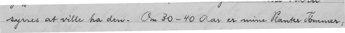synes at ville ha den. Om 30-40 Aar er mine Planter Tømmer,
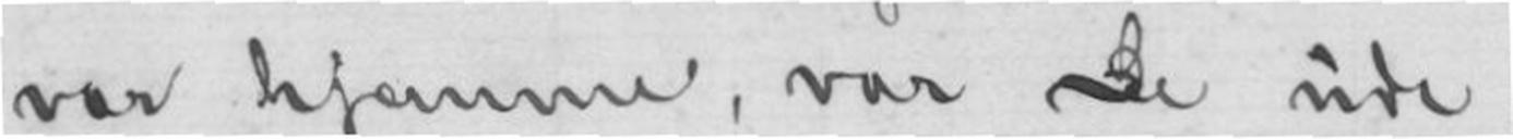var hjemme, var De ude,
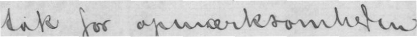tak for opmærksomheden
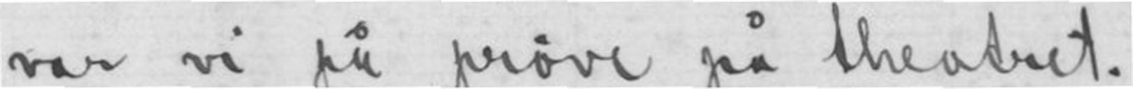var vi på prøve på theatret.
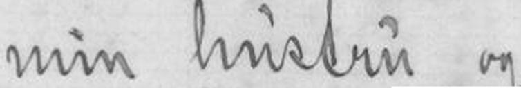min hustru og
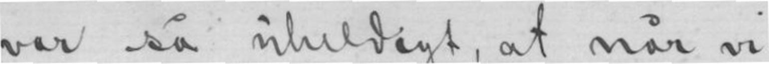var så uheldigt, at når vi
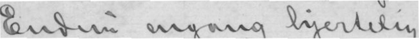Endnu engang hjertelig
